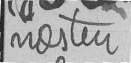næsten
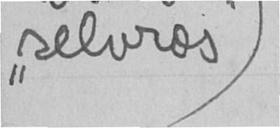"selvros")
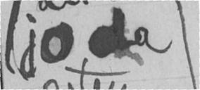jo sa
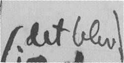(det blev
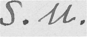S.U.
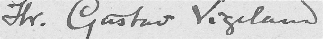Hr. Gustav Vigeland
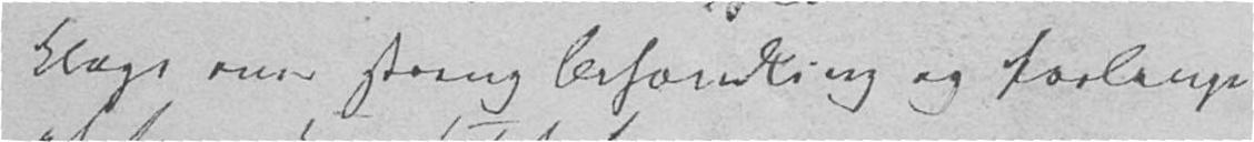klage over streng Behandling og forlange
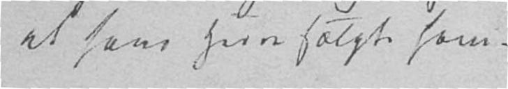at hans Herre solgte ham.
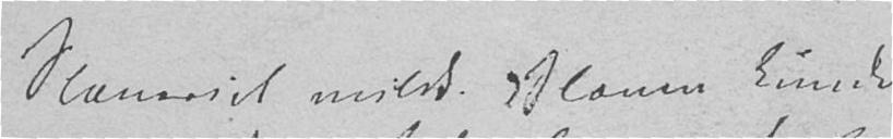Slaveriet mildt. Slaven kunde
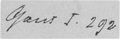Gans I. 292
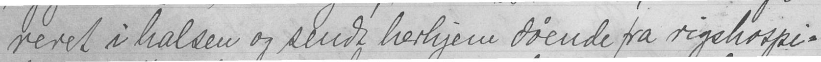reret i halsen og sendt herhjem døende fra rigshospi-
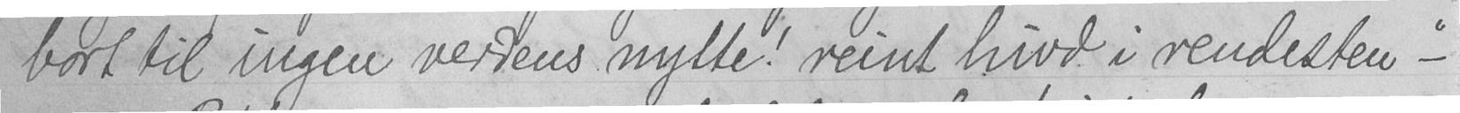bort til ingen verdens nytte! reint hivd i rendesten - "
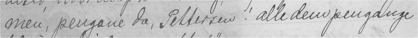men, pengane da, Pettersen! alle dem pengange
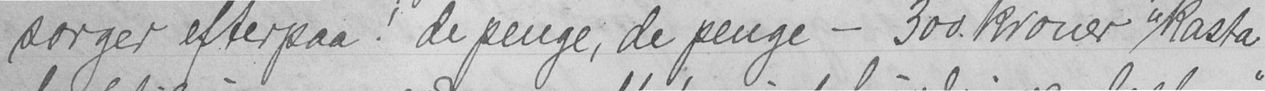sorger efterpaa! de penge, de penge - 300 kroner "kasta
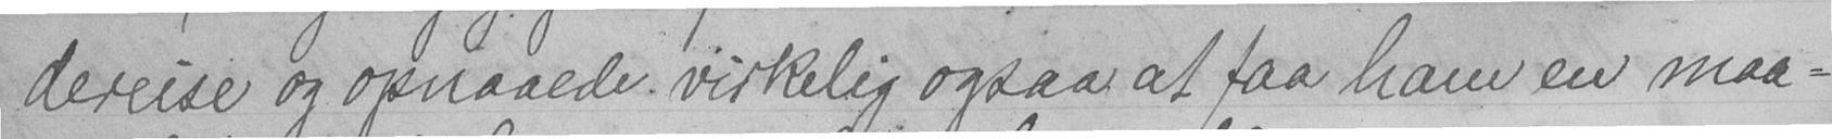dereise og opnaaede virkelig ogsaa at faa ham en maa-
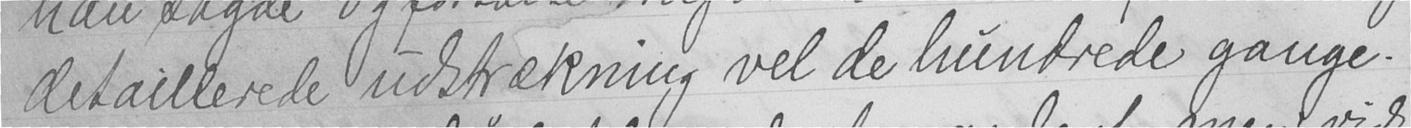detaillerede udstrækning vel de hundrede gange.
